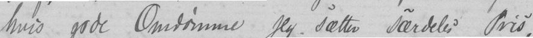hvis gode Omdømme jeg sætter særdeles Pris,
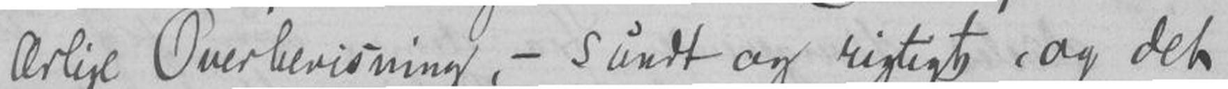Ærlige overbevisnin,- sandt og rigtigt, og det
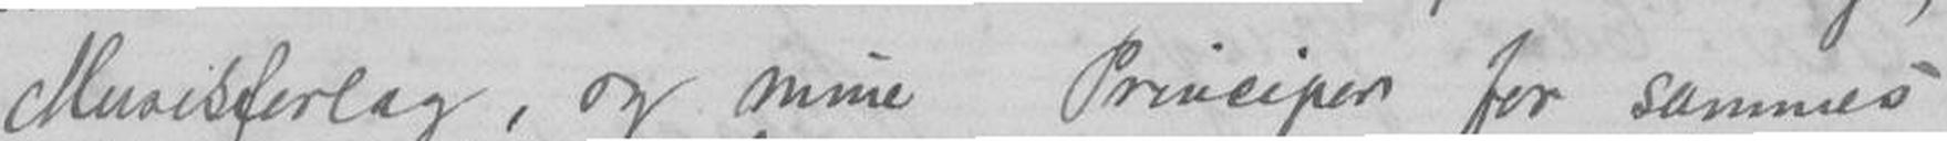musikforlag, og mine Principer for samme
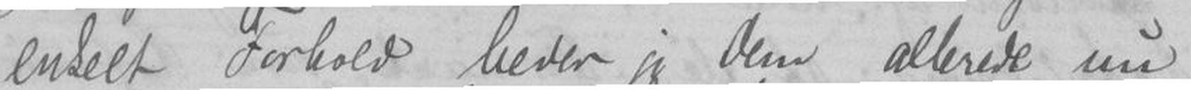enkelt Forhold beder jeg dem allerede nu
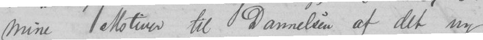mine Motiver til Dannelsen af det nye
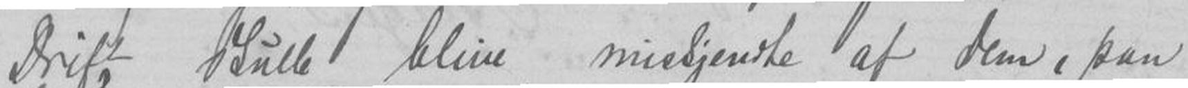Drift skulle blive miskjendte af Dem, paa
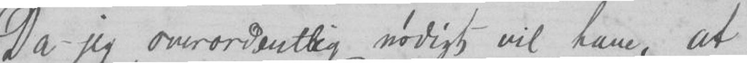Da jeg overordentlig mødigt vil have, at
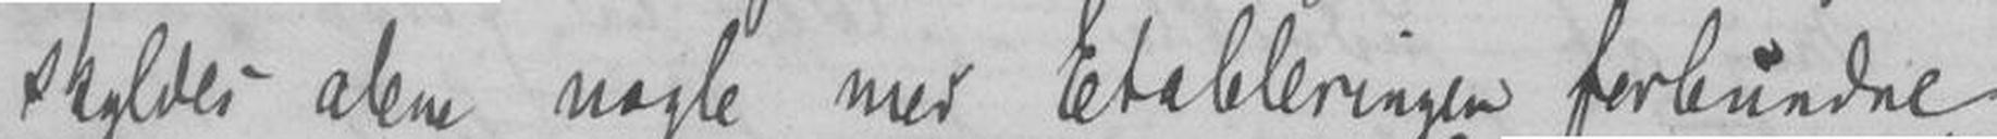skyldes alene nogle med Etableringer forbundne
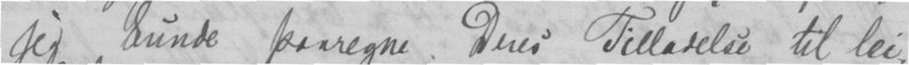jeg kunde paaregne deres Tilldelse til lei-
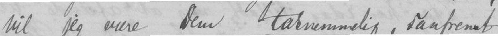vil jeg være Dem taknemlig, Saafremt
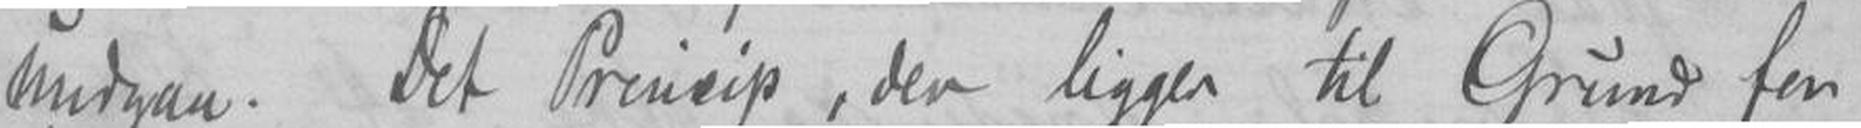Undgaa. Det princip, det ligger til Grund for
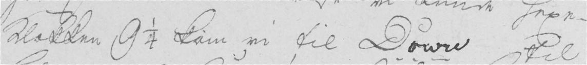Klokken 9 1/4 kom vi til Dovre til
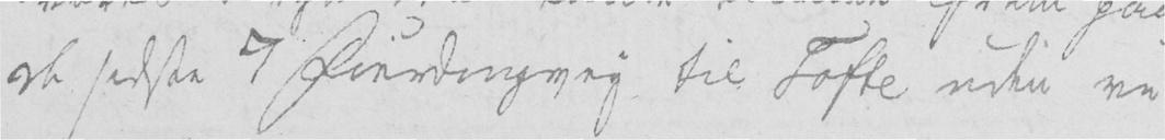de sidste 7 Fierdingvey til Tofte uden vi
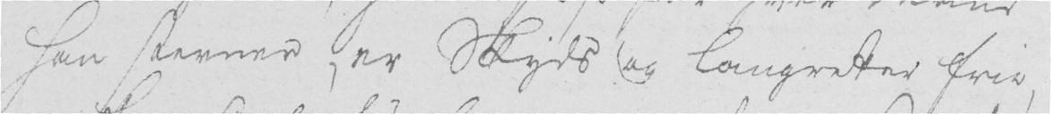han stevner, er Skyds og laugreter frie,
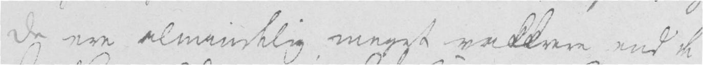de ere almindelig meget vakkrere end de
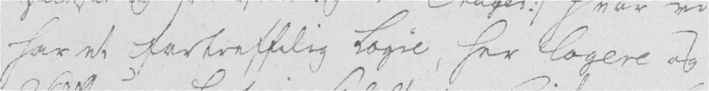har et fortreffelig Logie, her logere og
